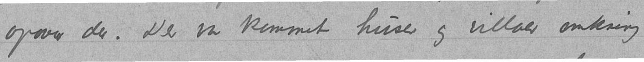opover der. Der var kommet huser og villaer omkring
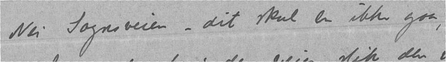Nei Sognsveien – dit skal en ikke gaa,
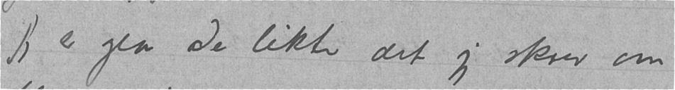Jeg er gla De likte det jeg skrev om
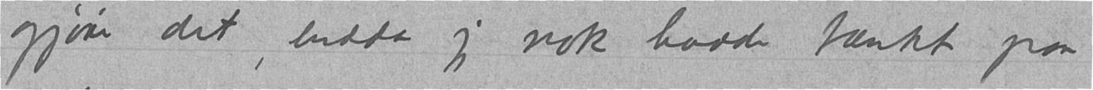gjøre det, endda jeg nok hadde tænkt paa
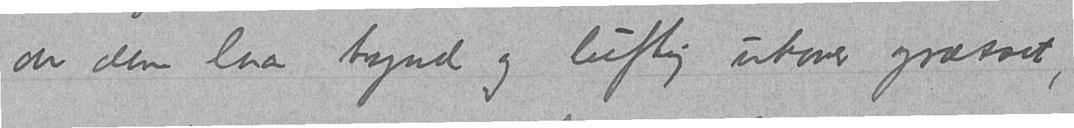av dem laa trynd og luftig uhover græsset,
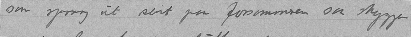som sprang ut sent paa forsommeren saa skyggen
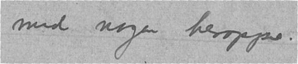med nogen heroppe.
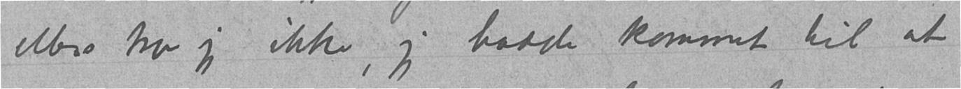ellers tror jeg ikke, jeg hadde kommet til at
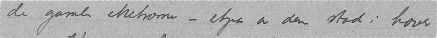de gamle eketrærne – etpar av dem stod i haver
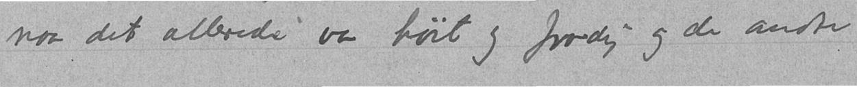naar det allerede var høit og frodig og de andre
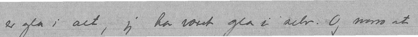er gla i alt, jeg har været gla i selv. Og morro at
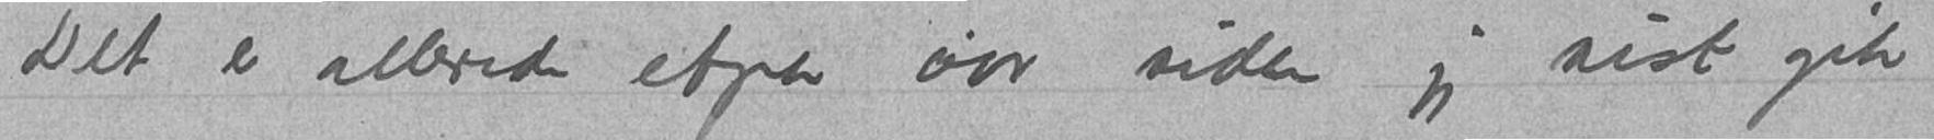Det er allerede etpar aar siden jeg sist gik
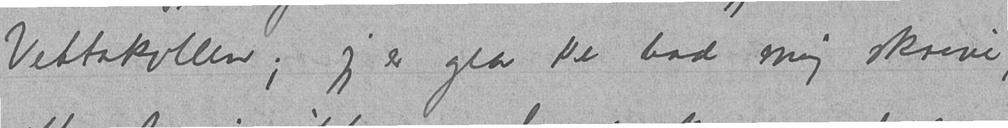Vettakollen; jeg er gla De bad mig skrive
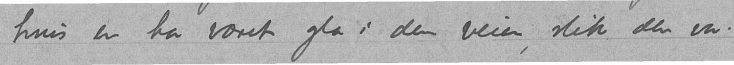hvis en har været gla i den veien slik den var.
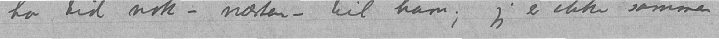ha tid nok – næsten – til ham; jeg er ikke sammen
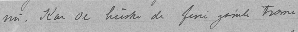nu. Kan de huske de fine gamle trærne
